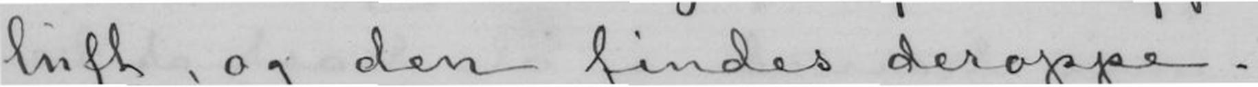luft, og den findes deroppe.
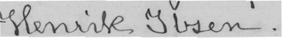Henrik Ibsen.
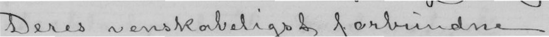Deres venskabeligst forbundne
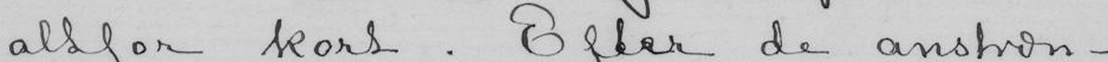altfor kort. Efter de anstræn-
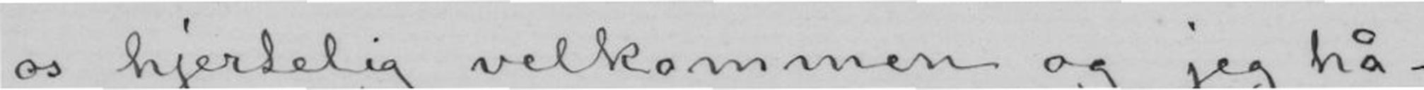os hjertelig velkommen og jeg hå-
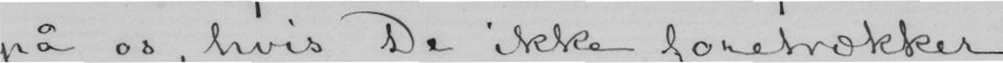på os, hvis De ikke foretrækker
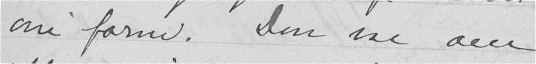om form. Don var om
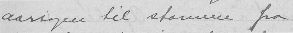aarsagen til stormen fra
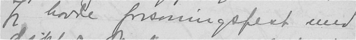Jeg havde forsoningsfest med
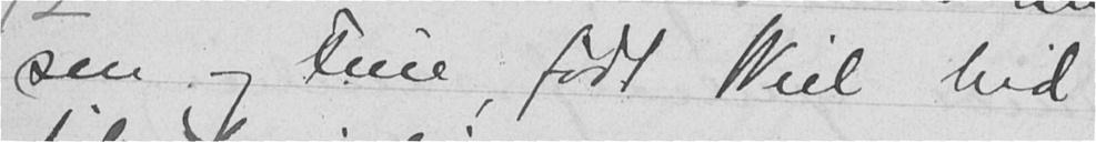sen og Frue, født Wiel hid
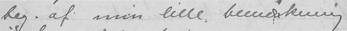beg. af min lille bemærkning
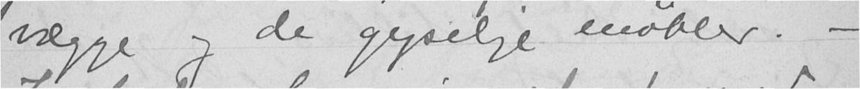vægge og de gyselige møbler. -
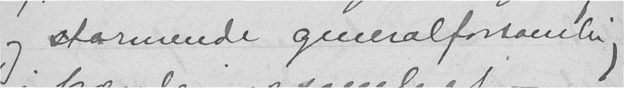og stormende generalforsamling
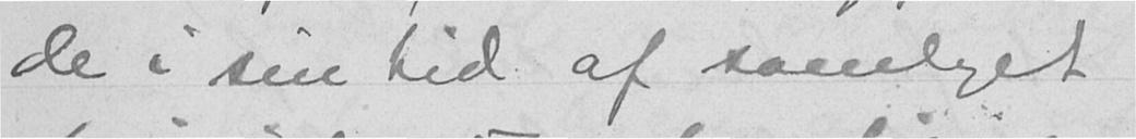de i sin tid af samlaget
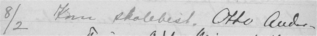8/2 Kom skolebest. Otto Ander-
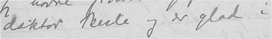doktor Berle og er glad i
